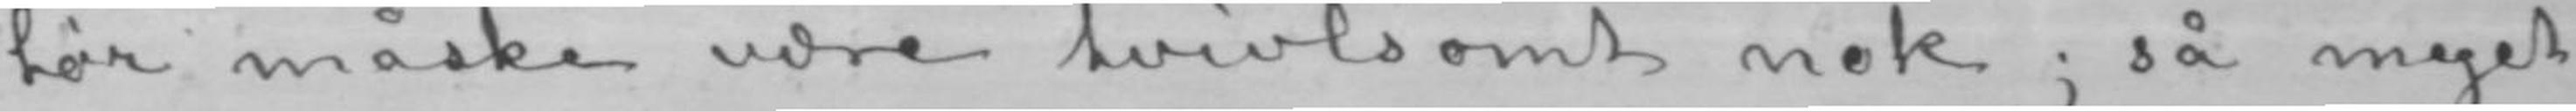tør måske være tvivlsomt nok; så meget
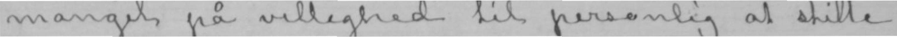mangel på villighed til personlig at stille
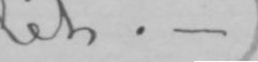let.-
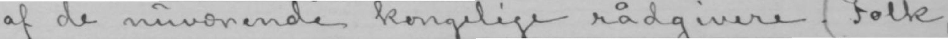af de nuværende kongelige rådgivere. Folk,
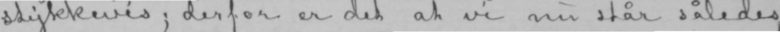stykkevis; derfor er det at vi nu står således,
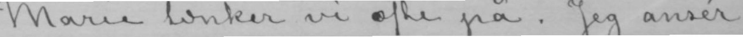Marie tænker vi ofte på. Jeg ansér
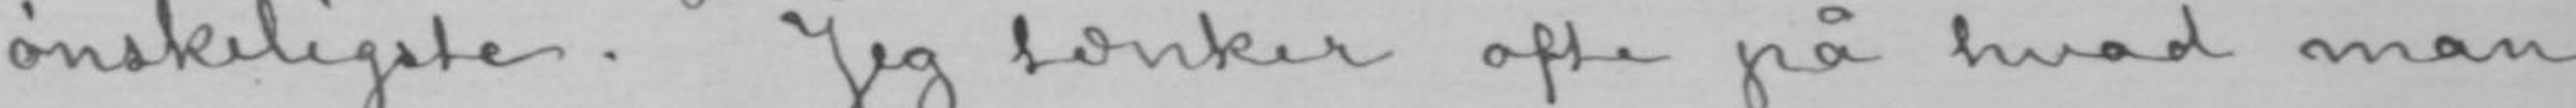ønskeligste. Jeg tænker ofte på hvad man
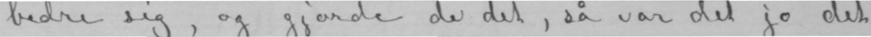bedre sig, og gjorde de det, så var det jo det
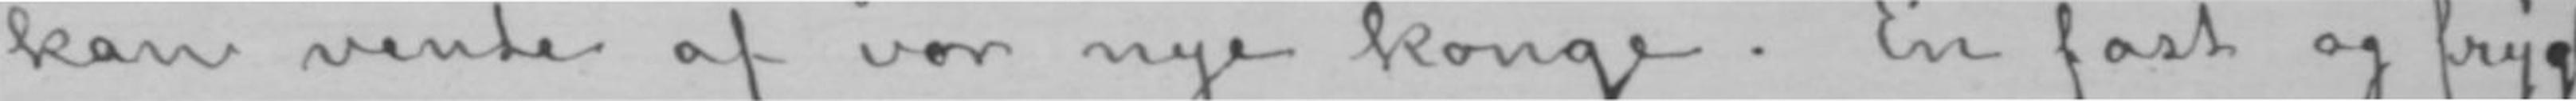kan vente af vor nye konge. En fast og frygt-
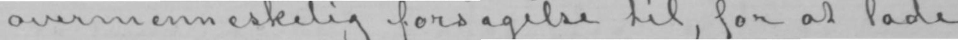overmenneskelig forsagelse til, for at lade
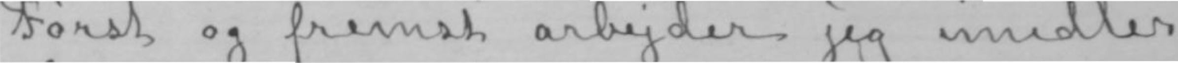Først og fremst arbejder jeg imidler-
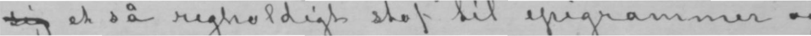sig et så righoldigt stof til epigrammer og
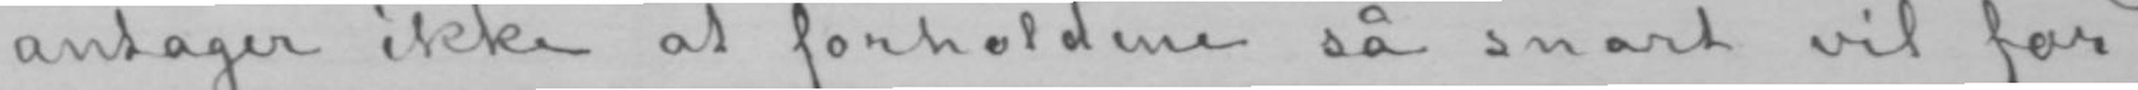antager ikke at forholdene så snart vil for-
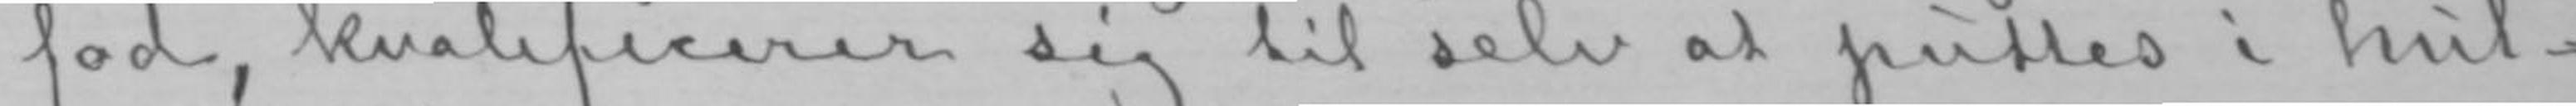fod, kvalificerer sig til selv at puttes i hul-
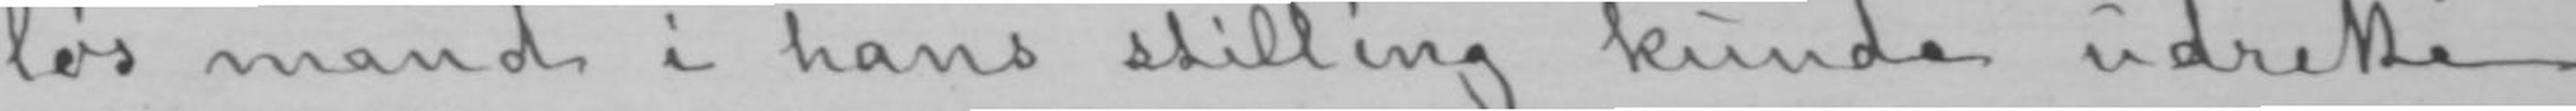løs mand i hans stilling kunde udrette
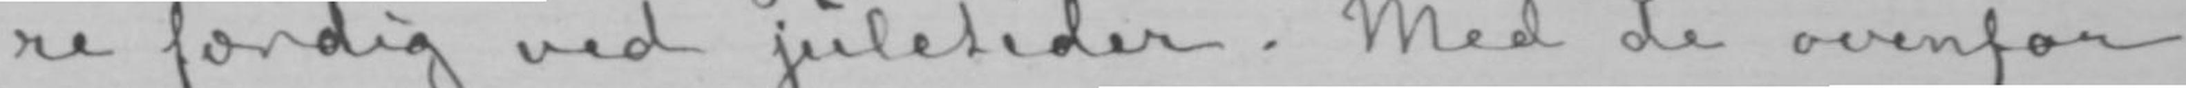re færdig ved juletider. Med de ovenfor
